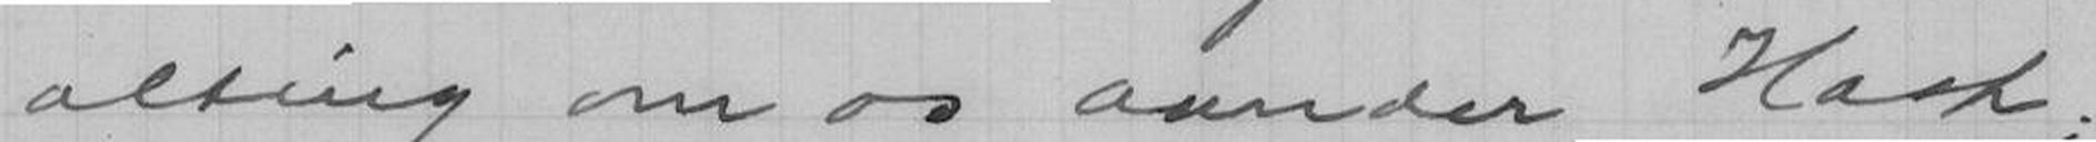alting om os aander Hast;
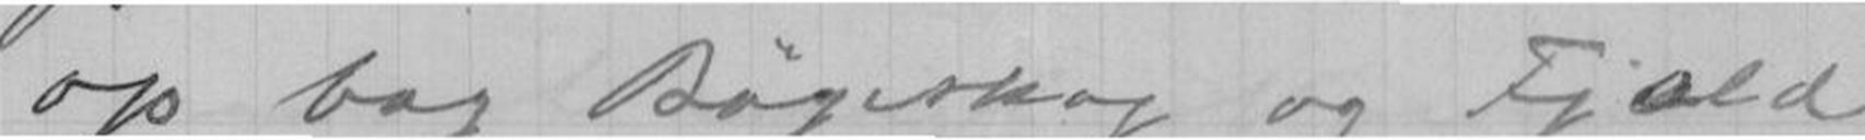op bag Bøgeskog og Fjæld
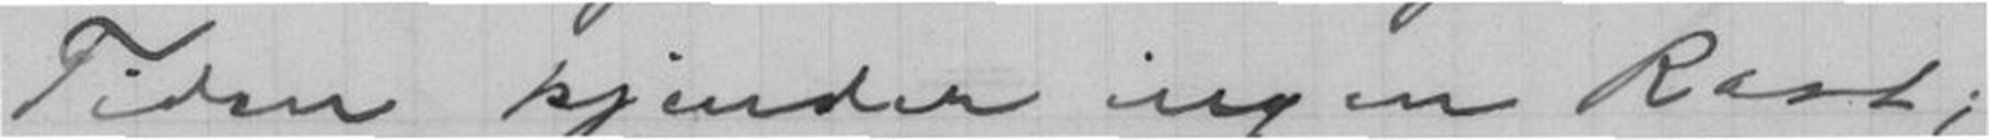Tiden kjender ingen Rast;
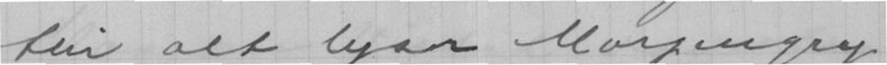thi alt lyser Morgengry
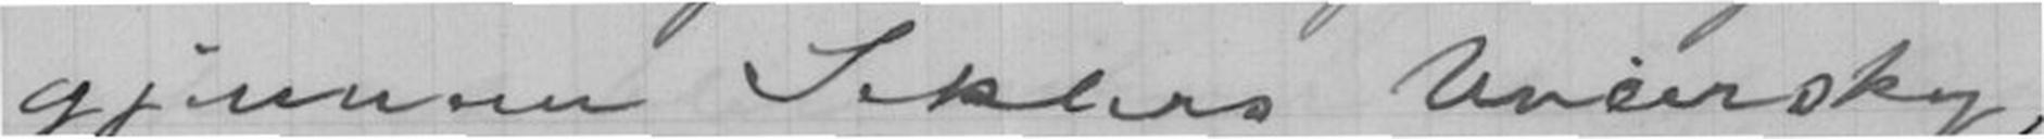gjennem Sekters Uveirsky;
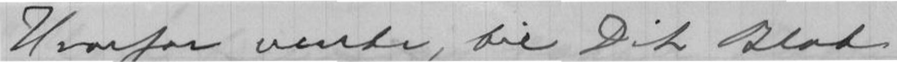Hvorfor vente, til Dit Blod
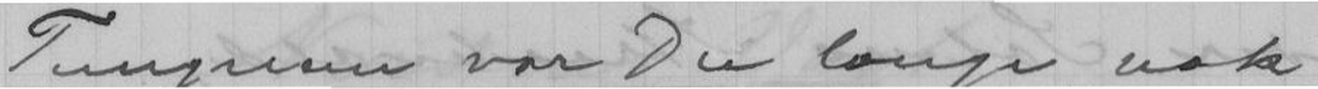Tungnem var Du længe nok!
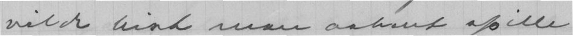vilde hist man aabent spille
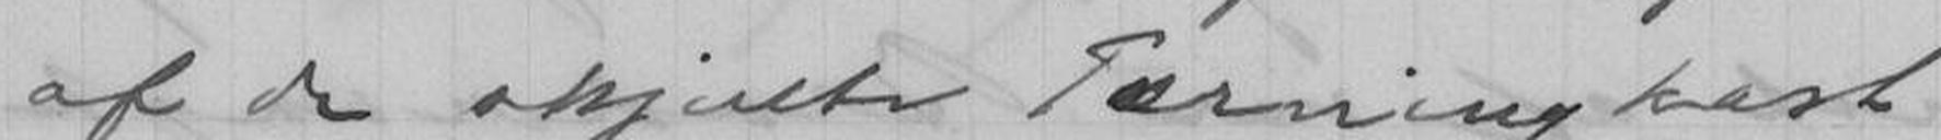af de skjulte Tærningkast?
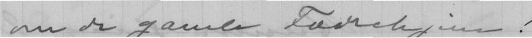om de gamle Fædrehjem!
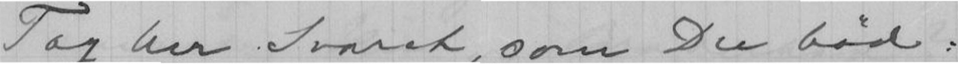Tag her Svaret, som Du bød:
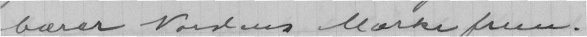bærer Nordens Mærke frem!
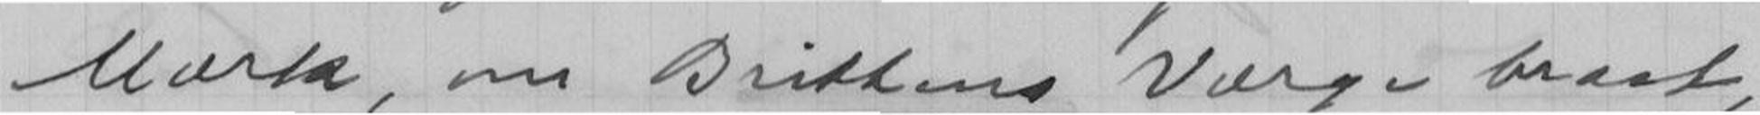Mærk, om Brikkens Værge brast,
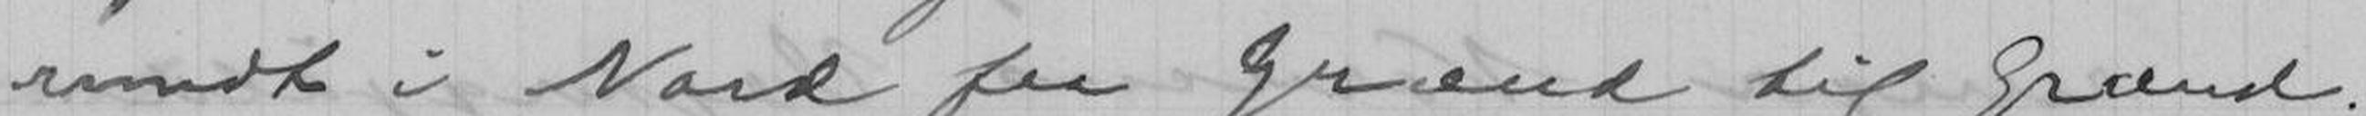rundt i Nord fra Grænd til Grænd!
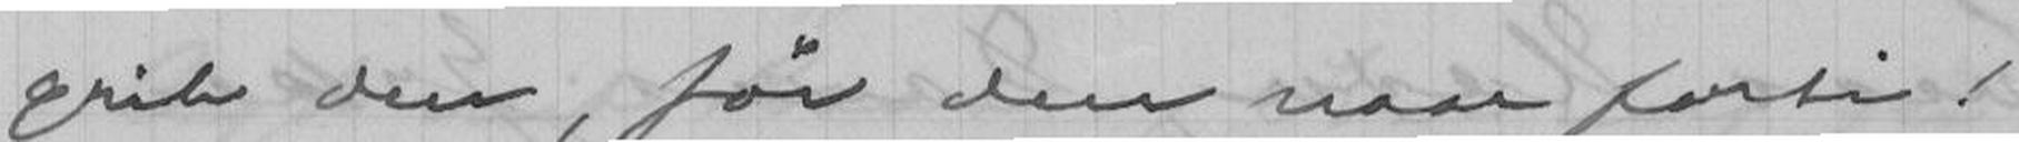grib den, før den naar forbi!
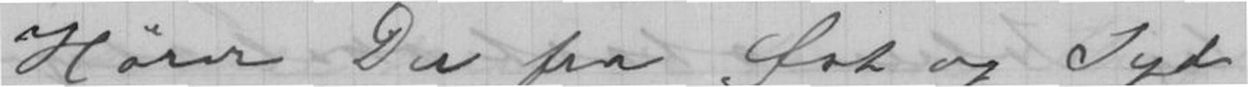Hører Du fra Øst og Syd
